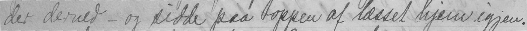der derned - og sidde paa toppen af læsset hjem igjen.
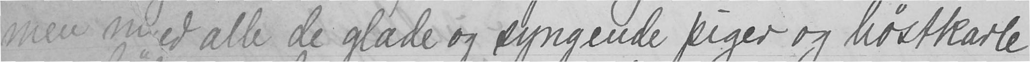men med alle de glade og syngende piger og høstkarle
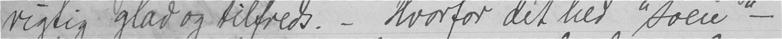rigtig glad og tilfreds. - Hvorfor det hed "søen" -
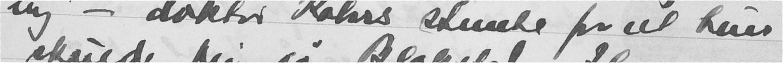mig - doktor Kahrs stemte for at hun
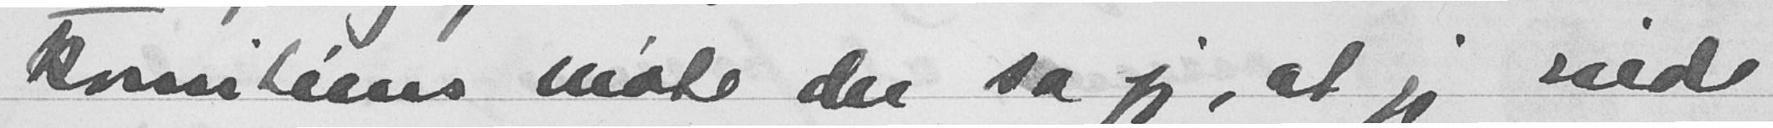Familiens møte der sa jeg, at jeg vilde
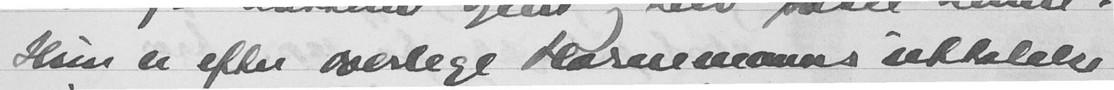Hun er efter overlege Hasneneannus uttalelser
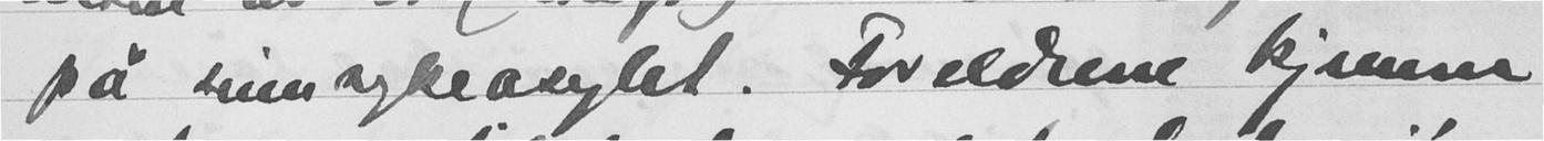på sinnsykasylet. Foreldrene kjenner
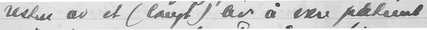resten av et (langt) liv å være pasient
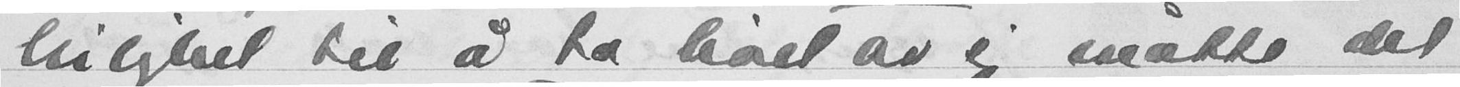leilighet til å ta livet av sig måtte det
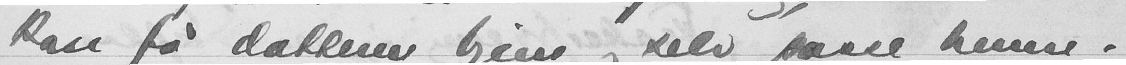kan få datteren hjem og selv passe henne.
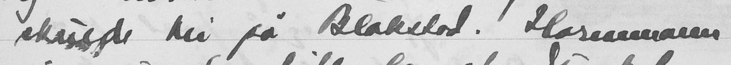skulde bli på Blakstad. Harmmales
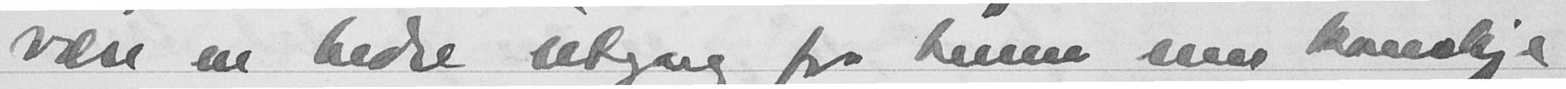være en bedre utgang for henne enn kanskje
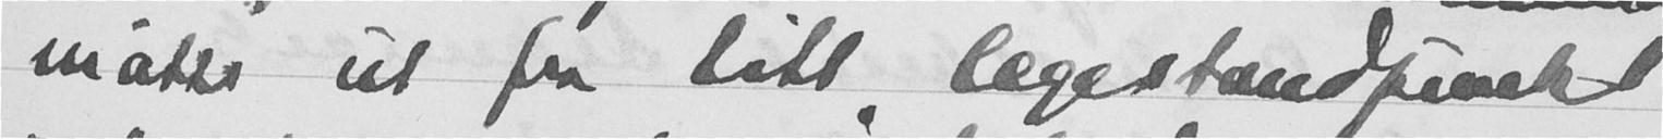måtte ut fra sitt legestandpunkt
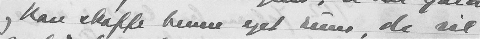og kan skaffe henne eget rum, de vil
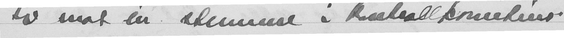to mot en stemme i kontrollkomiteen.
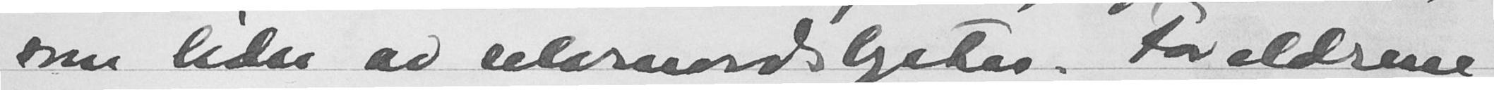som lider av selvmordslyster. Foreldrene
